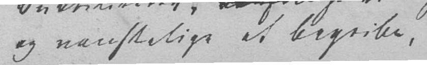og vanskelige at begribe,
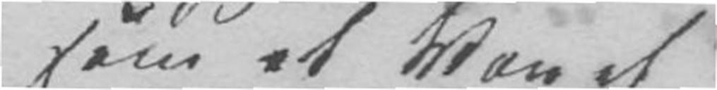som et Van af
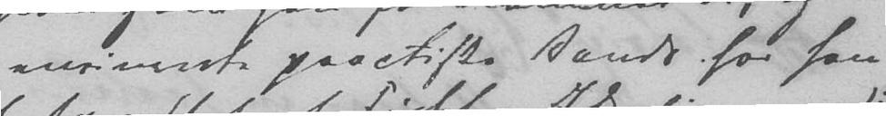eminente practiske Sands har han
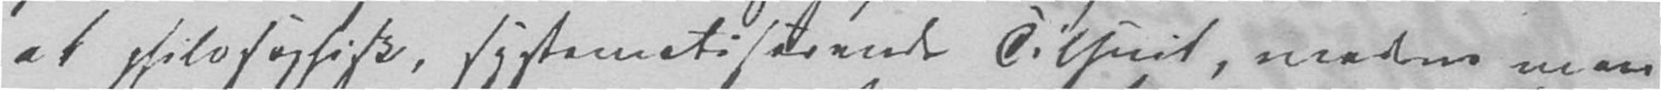et philosopisk, systematiserende Tilsnit, medens man
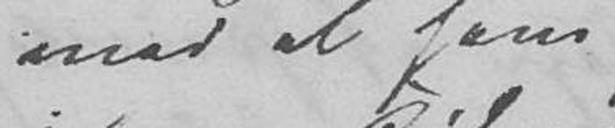med al hans
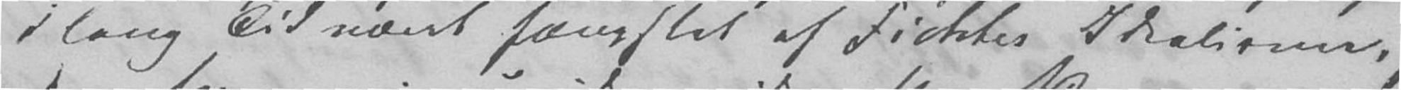i lang Tid været fængslet af Fichtes Idealisme,
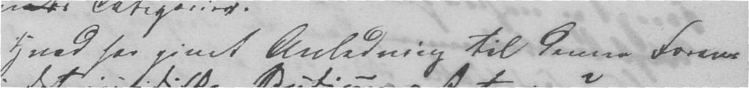Hvad har givet Anledning til denne Foran-
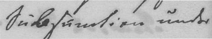Subsumtion under
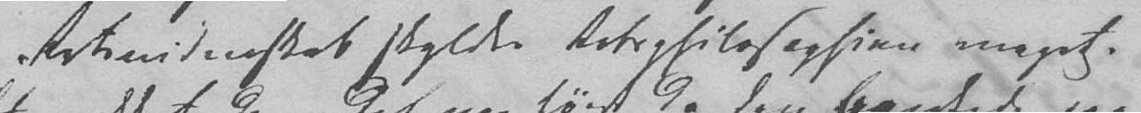Retsvidenskab skylder Retsphilosophien meget.
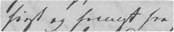først og fremst fra
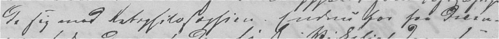de sig med Retsphilosophien. Endnu for faa Decen-
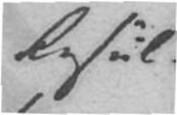Resul-
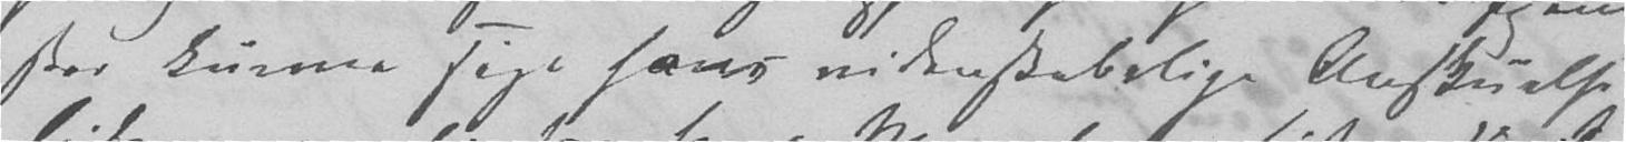ester kunne sige hans videnskabelige Anskuelse
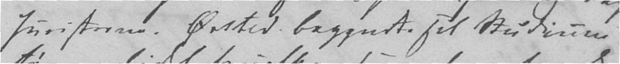Juristerne. Ørsted begyndt sit Studium
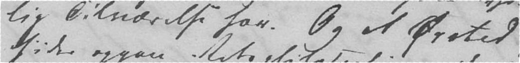lig Tilværelse har. Og at Ørsted
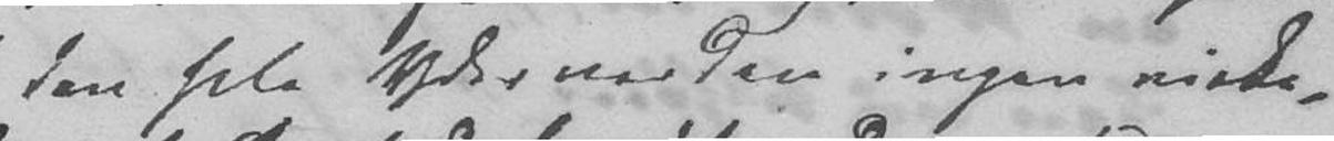den hele Yderverden ingen virke-
